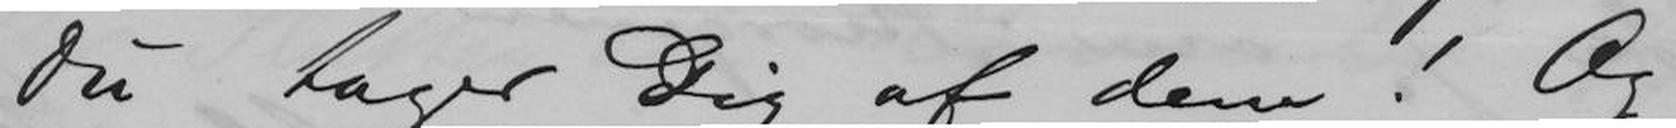du tager Dig af dem! Og
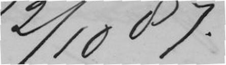22/10 87
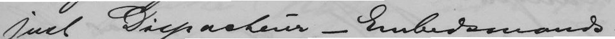just Dispacheur - Embedsmands -
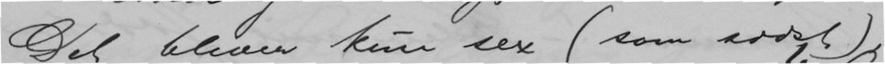Det bliver kun sex (som sidst)
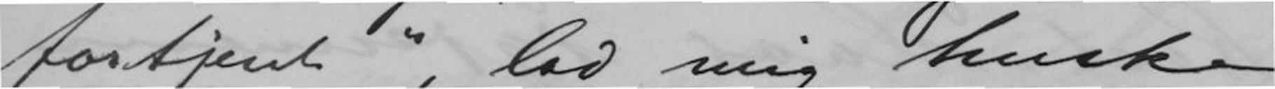fortjent", lad mig huske
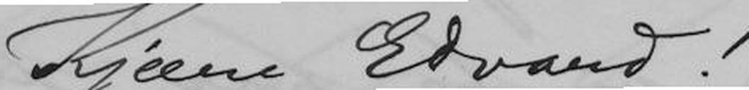Kjære Edvard!
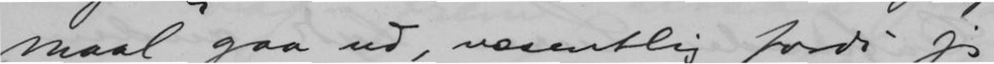maal gaa ud, væsentlig fordi jeg
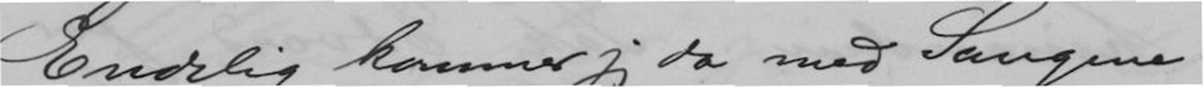Endelig kommer jeg da med Sangene.
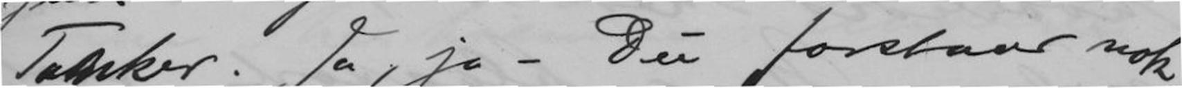Tanker. Ja, ja - Du forstaar nok.
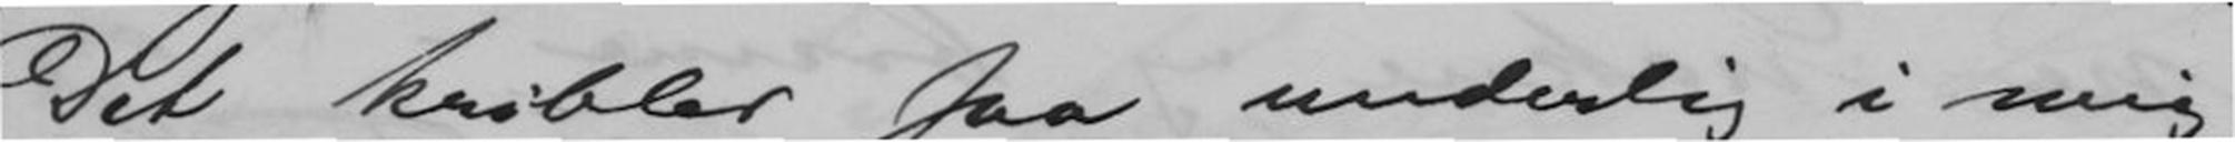Det kribler saa underlig i mig,
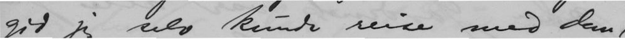gid jeg selv kunde reise med dem!
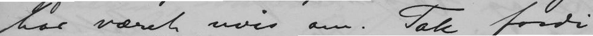har været uvis om. Tak fordi
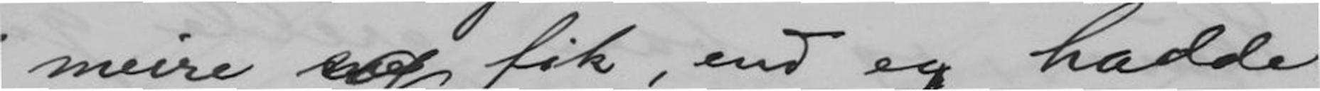"meire eg fik, end eg hadde
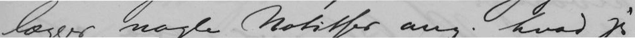lægger nogle Notitser ang. hvad jeg
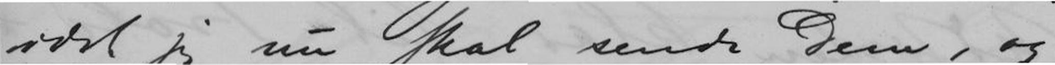idet jeg nu skal sende Dem, og
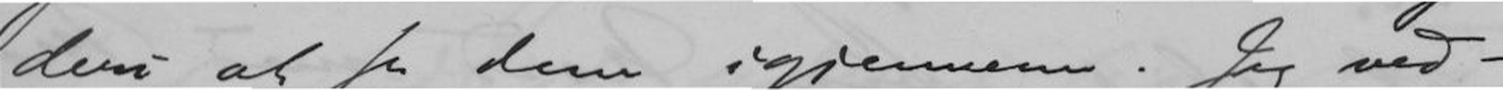deri at se dem igjennem. Jeg ved-
